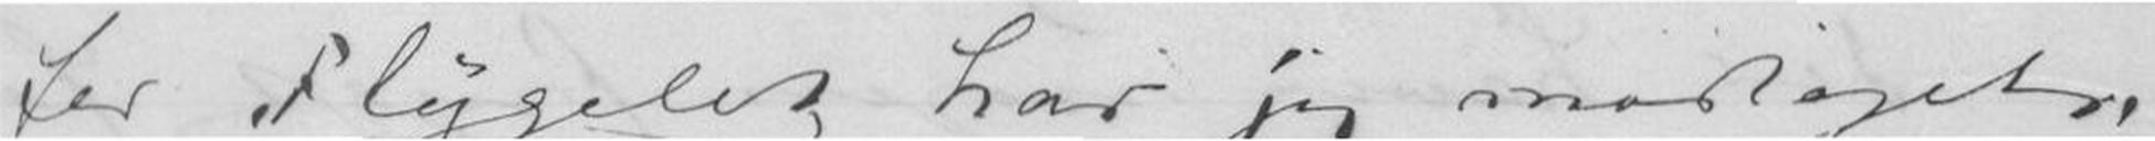for Flygelet har jeg modtaget,
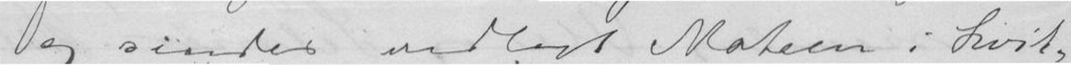og sender vedlagt Notaen i kvit-
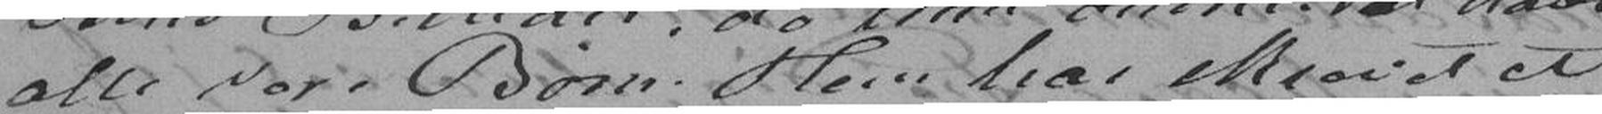alle vore Børn. Hun har skrevet et
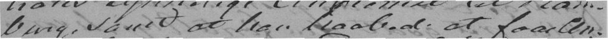burg, samt at han haabede at faae An-
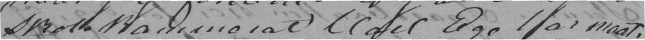Skolekammerat Carl Ege har maat-
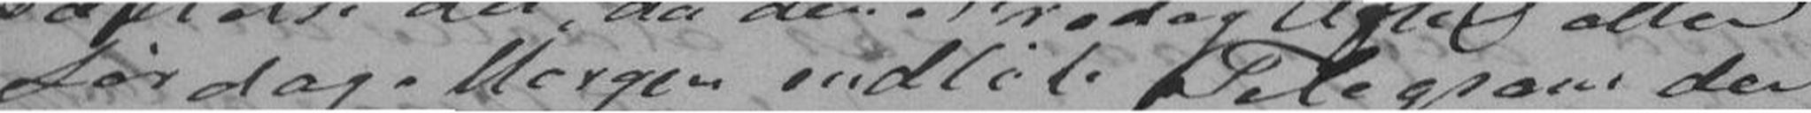Lørdag Morgen indløb Telegram der
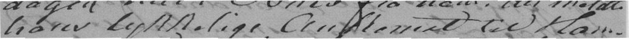hans lykkelige Ankomst til Ham-
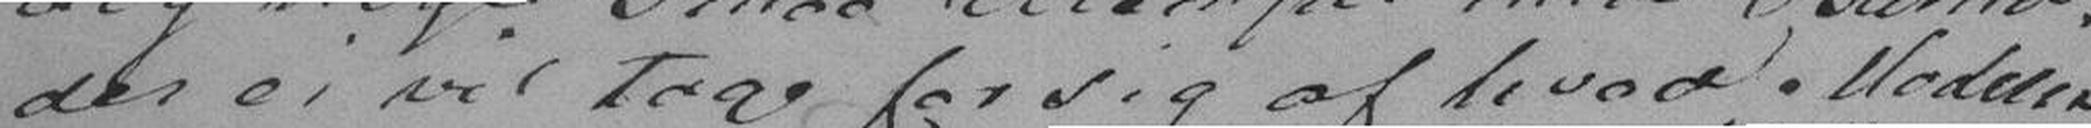der ei vil tage for sig af hvad Moderen
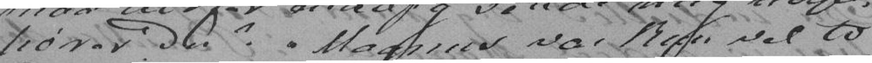hører Du. Magnus var kun vel to
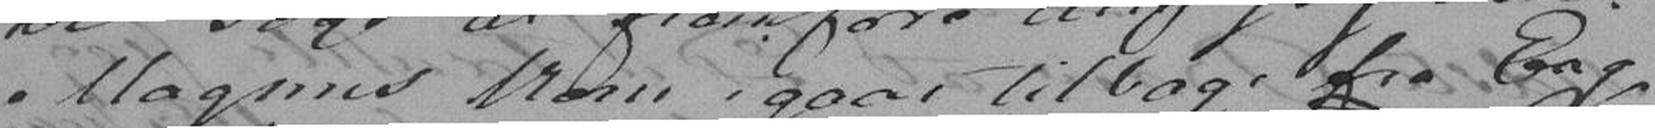Magnus kom igaar tilbage fra Eng-
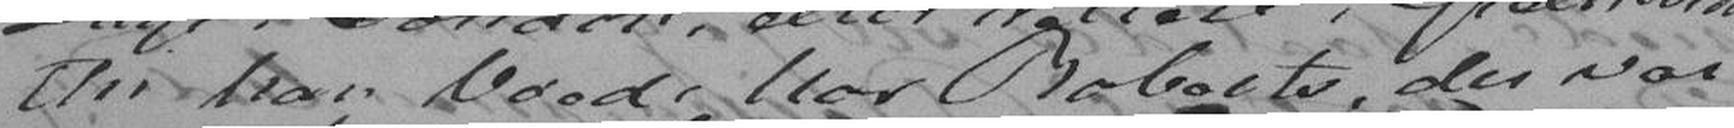thi han boede hos Roberts, der var
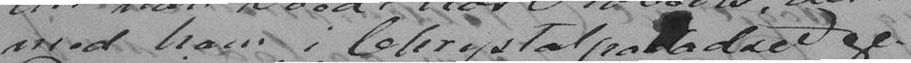med ham i Chrystalpalladset ogc -
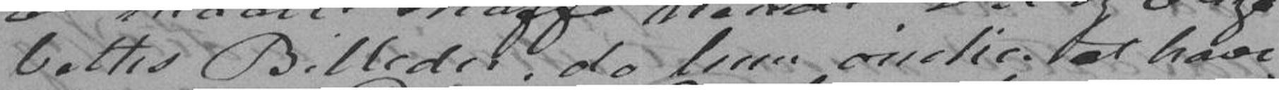beths Billede, da hun ønske at have
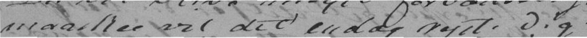maaskee vil det endog ryste Dig -
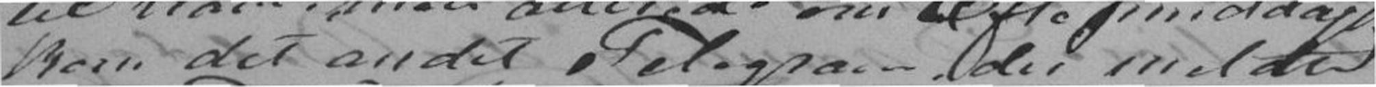kom det andet Telegram - der meldte
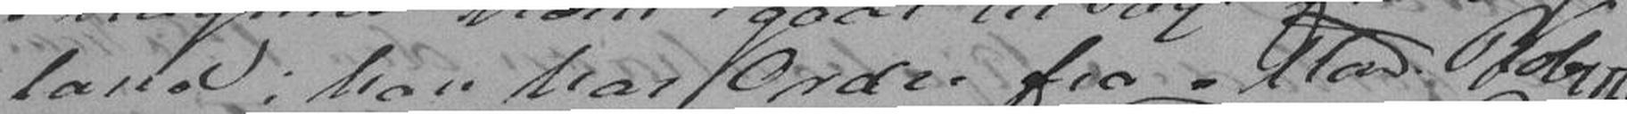land; han har Ordre fra Mad. Robert,
